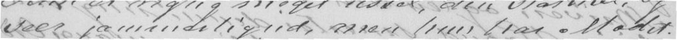seer jammerlig ud, men hun har Modet.
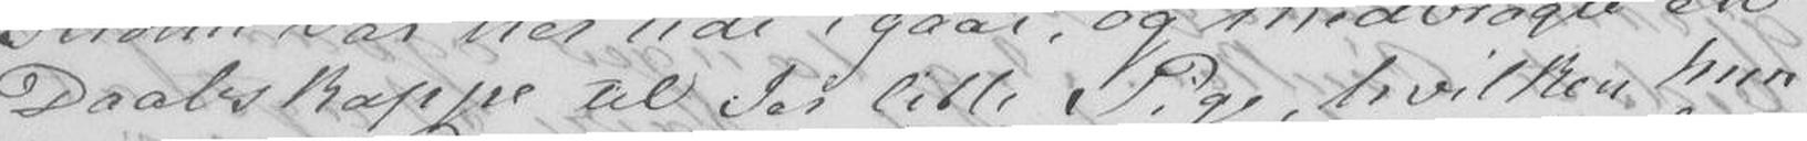Daabskappe til Jeg lille Pige, hvilken hun
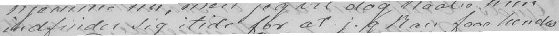indfinder sig itide for at jeg kan faae hendes
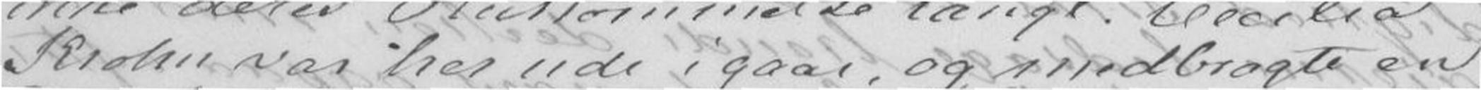Krohn var her ude igaar, og medbragte en
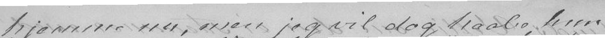hjemme nu, men jeg vil dog haabe hun
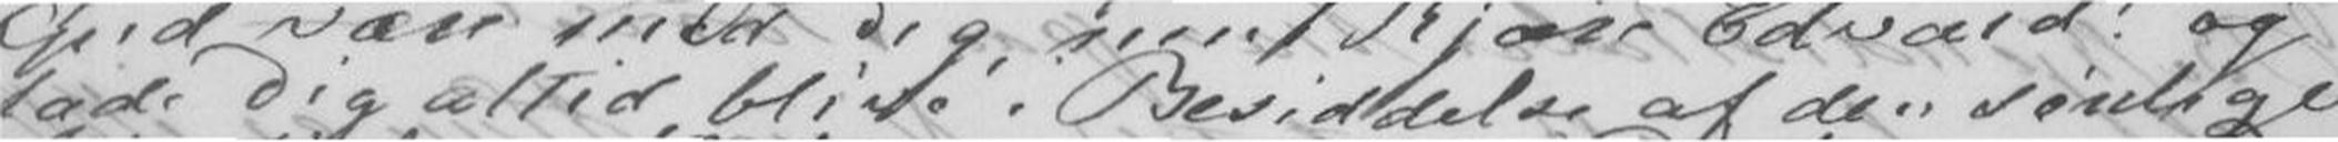lade Dig altid blive i Besiddelse af den sønlige
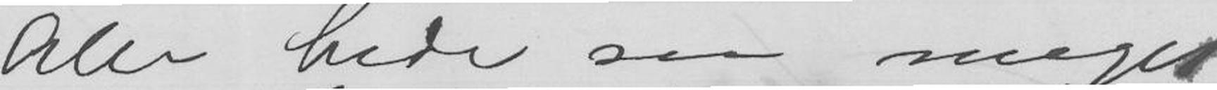Alle bede saa meget
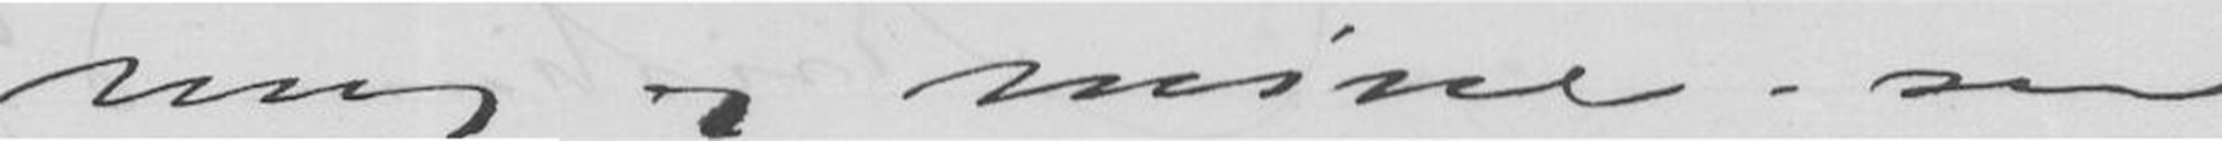mig og mine - som
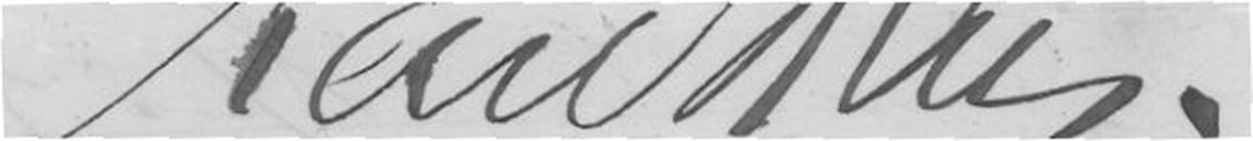Karl Hals
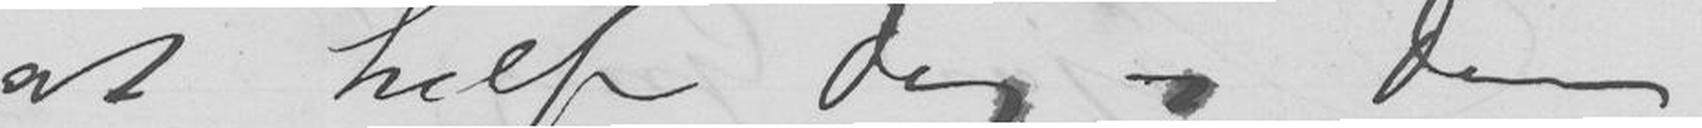at hilse Dig og Din
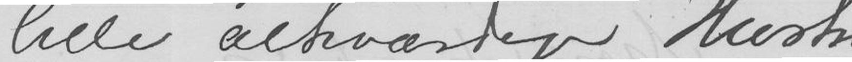lille ælkværdige Hustru
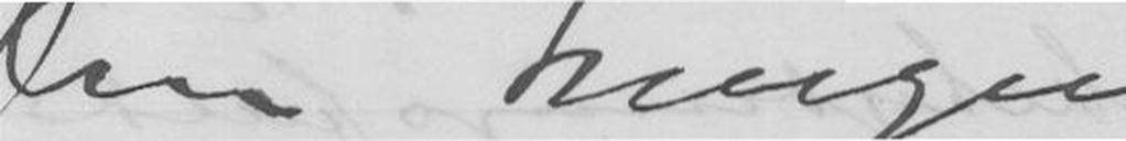Din hengivne
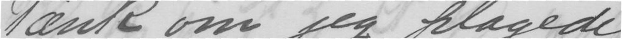Tænk om jeg plagede
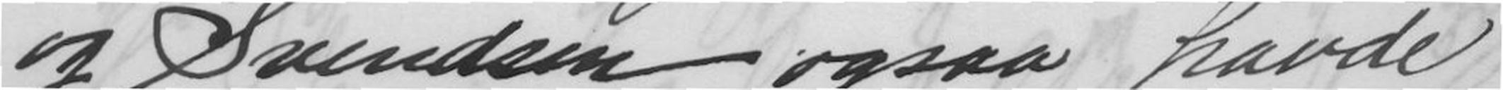og Svendsen ogsaa havde
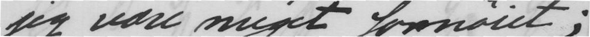jeg være meget fornøiet;
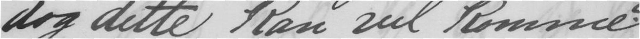dog dette kan vel komme?
